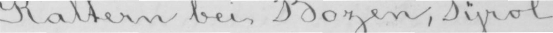Kaltern bei Bozen, Tyrol,
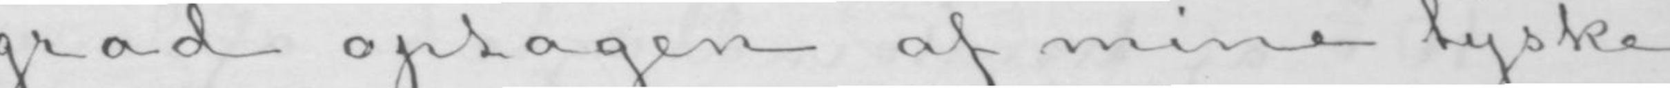grad optagen af mine tyske
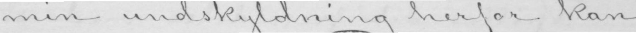min undskyldning herfor kan
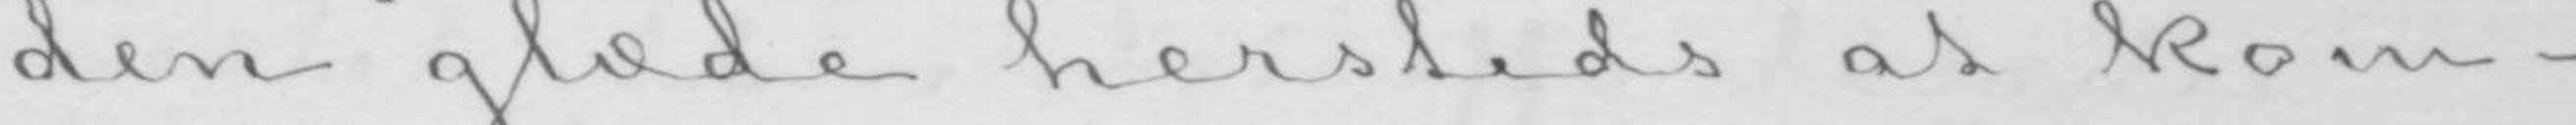den glæde hersteds at kom-
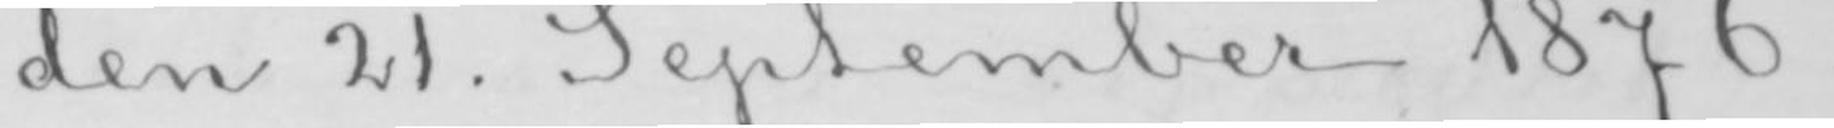den 21. September 1876.
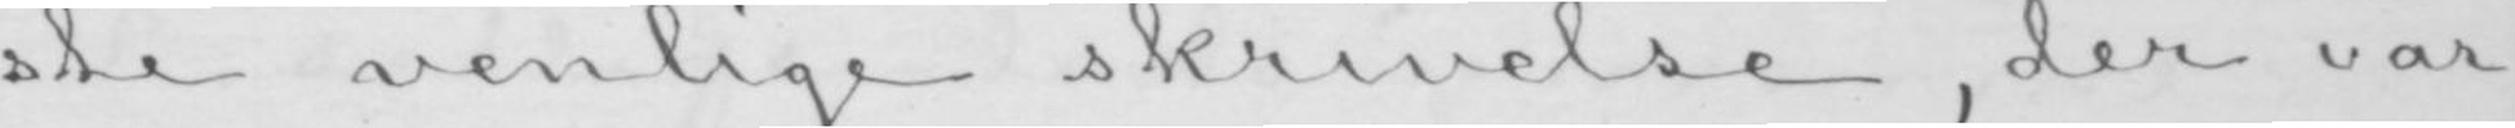ste venlige skrivelse, der var
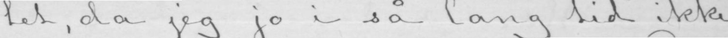tet, da jeg jo i så lang tid ikke
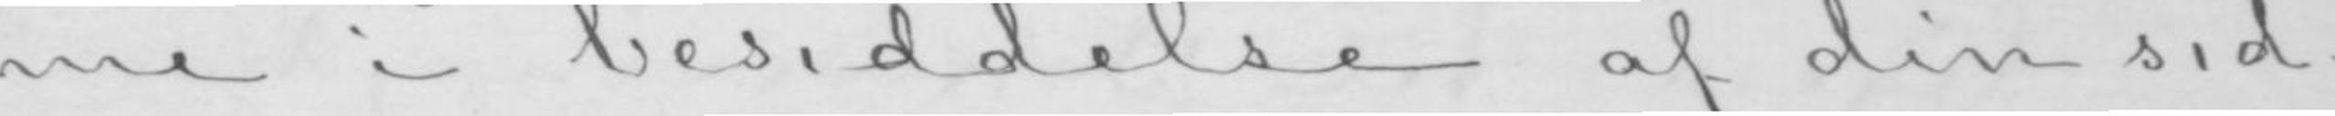me i besiddelse af din sid-
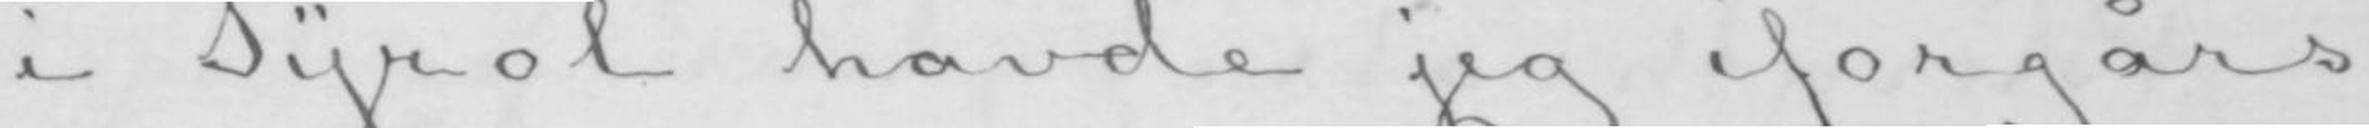i Tyrol havde jeg iforgårs
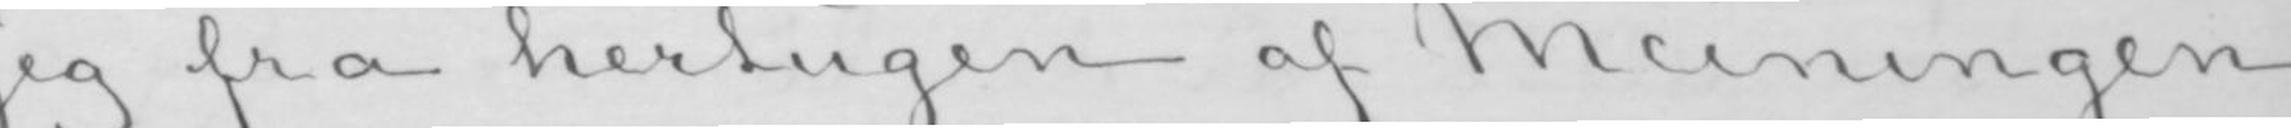jeg fra hertugen af Meiningen
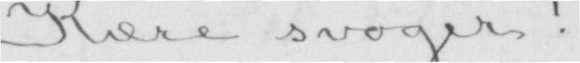Kære svoger!
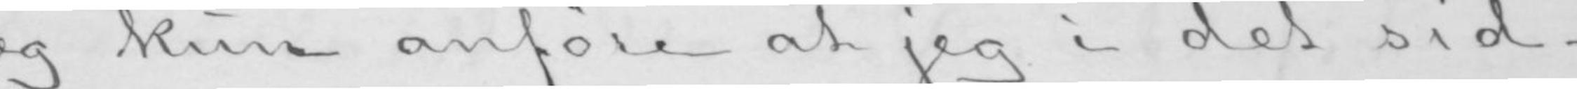jeg kun anføre at jeg i det sid-
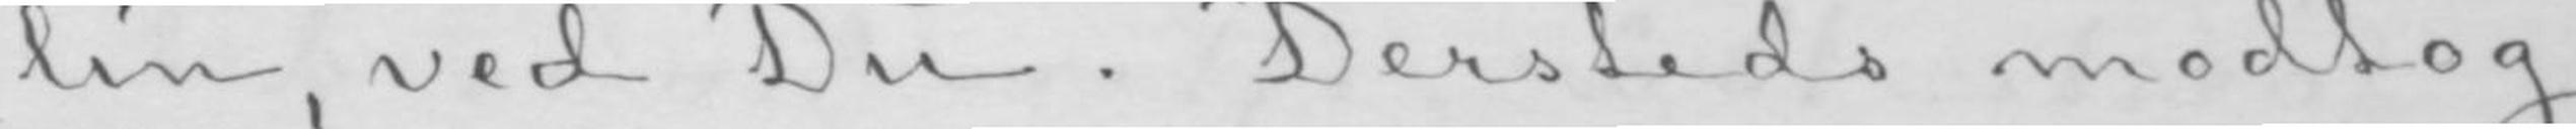lin, ved Du. Dersteds modtog
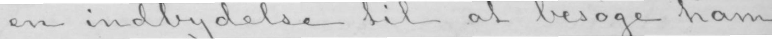en indbydelse til at besøge ham
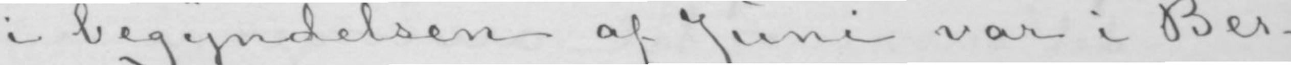i begyndelsen af Juni var i Ber-
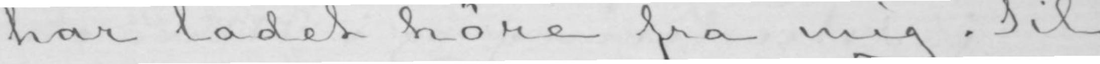har ladet høre fra mig. Til
